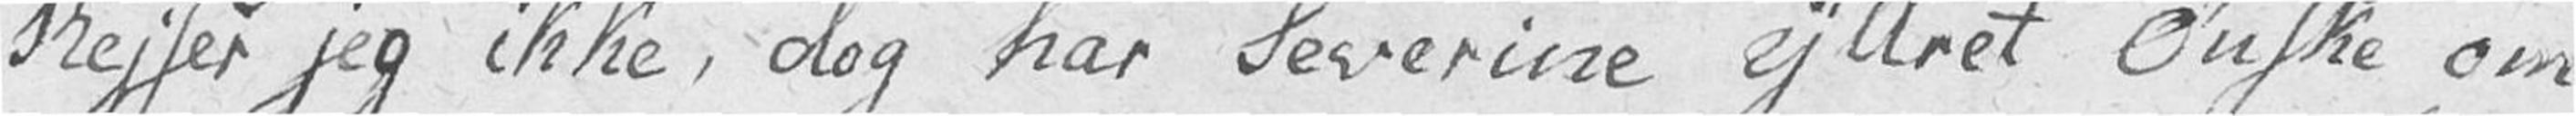Rejser jeg ikke, dog har Severine yttret Ønske om
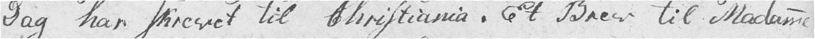Dag har skrevet til Christiania. Et Brev til Madamme
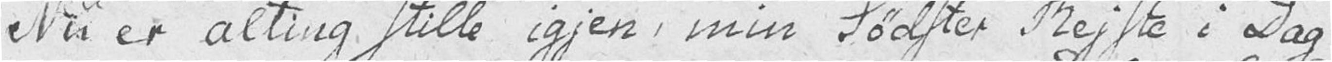Nu er alting stille igjen, min Sødster Rejste i Dag
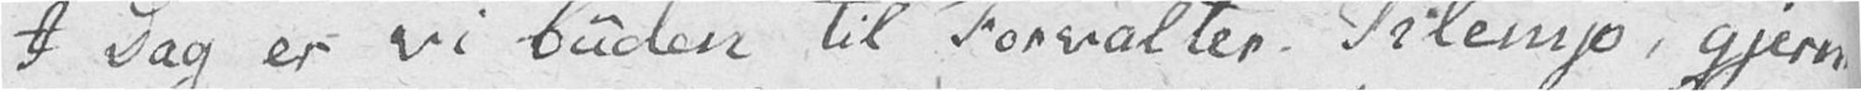I Dag er vi buden til Forvalter Klemp, gjern
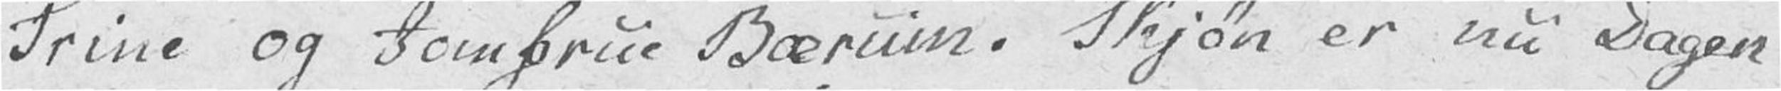Trine og Jomfrue Bærum. Skjøn er nu Dagen
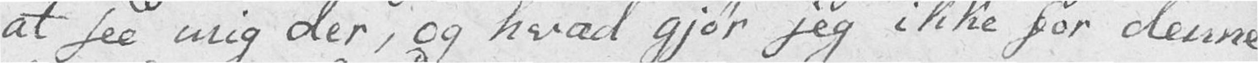at see mig der, og hvad gjør jeg ikke for denne
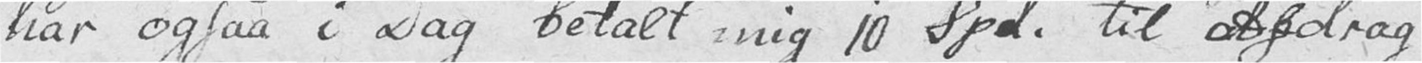har ogsaa i Dag betalt mig 10 Spd. til Afdrag
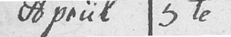Apriil 5te
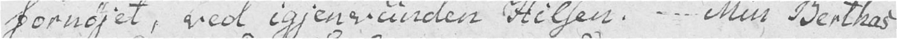fornøjet, ved igjenvunden Hilsen. – Min Berthas
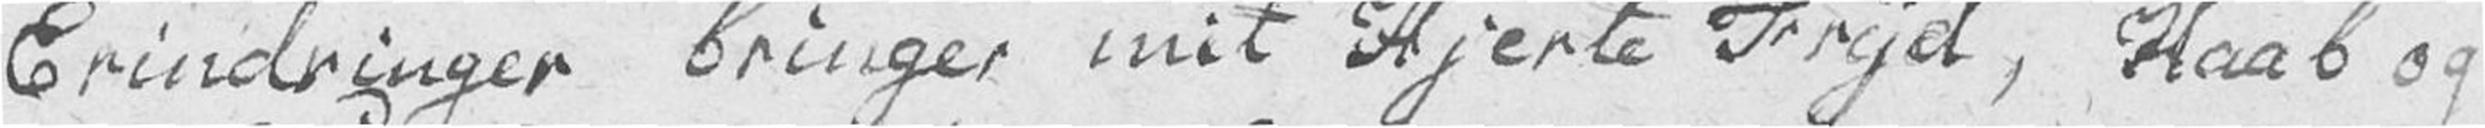Erindringer bringer mit Hjerte Fryd, Haab og
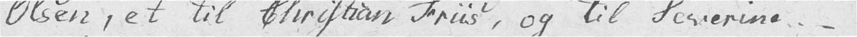Olsen, et til Christian Friis, og til Severine. –
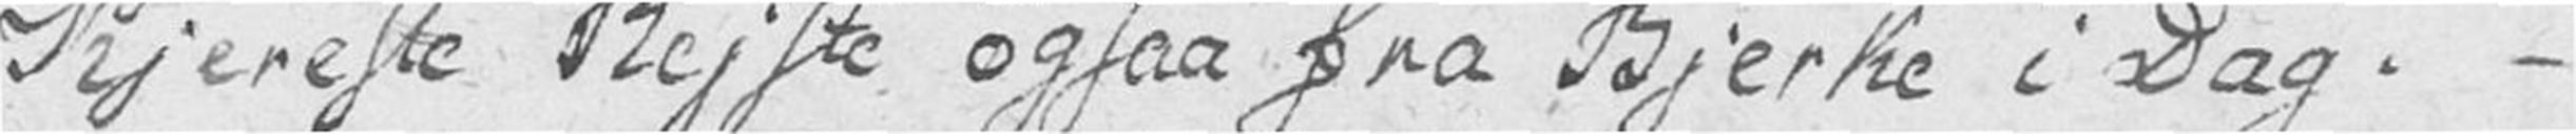Kjereste Rejste ogsaa fra Bjerke i Dag. –
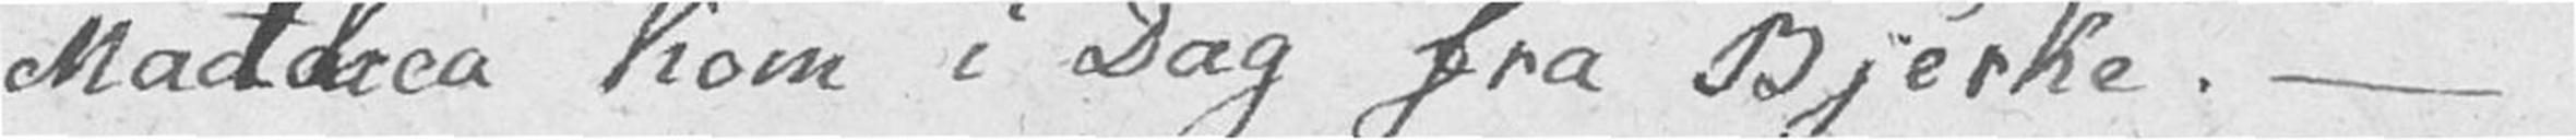Mathea kom i Dag fra Bjerke. –
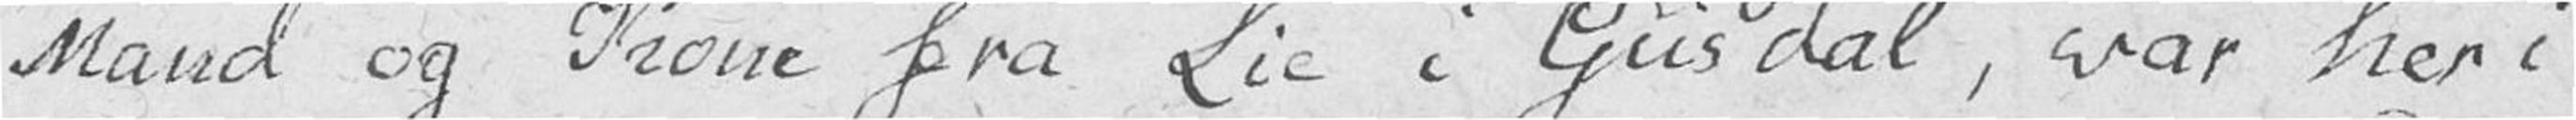Mand og Kone fra Lie i Gusdal, var her i
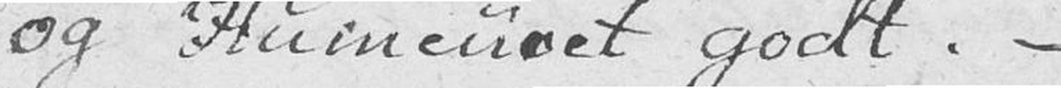og Humeuret godt. –
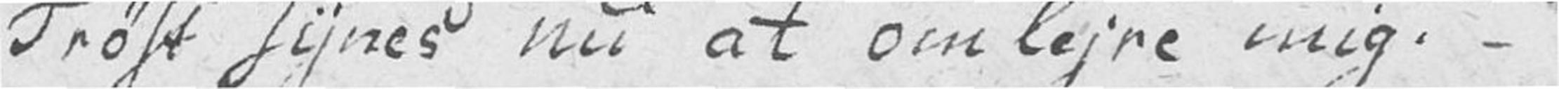Trøst synes nu at omlejre mig. –
